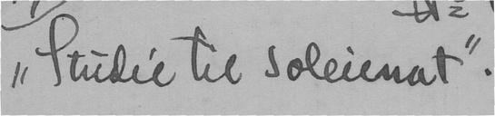"Studie til soleienat".
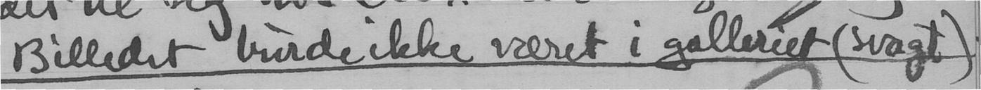Billedet burde ikke været i galleriet (svagt)
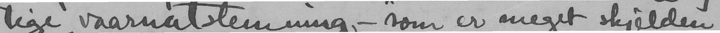tige vaarnatstemnig, - som er meget skjelden
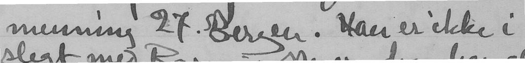menning 27 Bergen. Han er ikke i
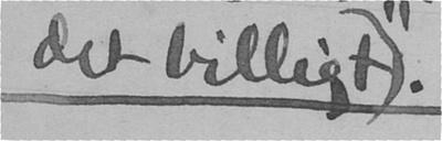det billigt).
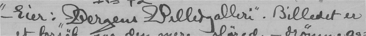- Eier: "Bergens Billedgalleri". Billedet er
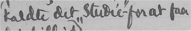kaldte det "studie" - for at faa
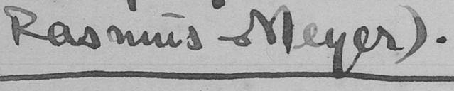Rasmus Meyer).
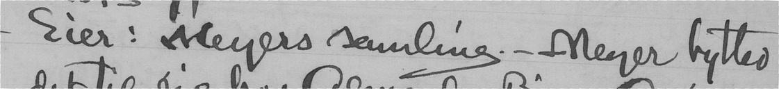Eier: Meyers samling. - Meyer bytted
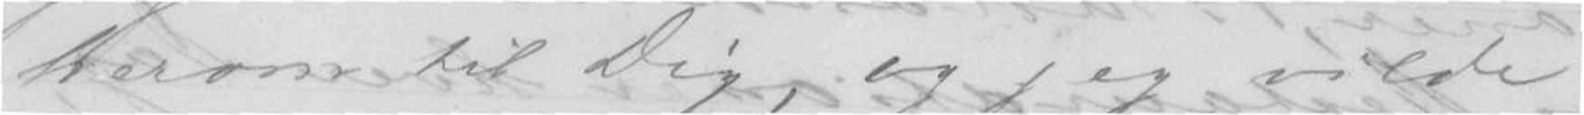herom til Dig, og jeg vilde
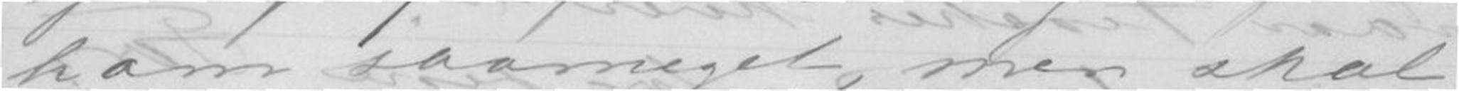ham saameget, men skal
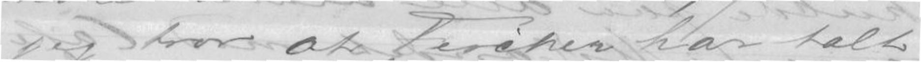jeg tror at Fischer har talt
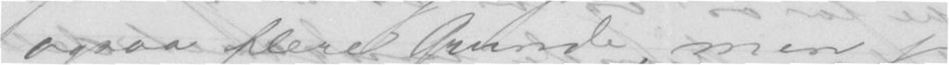ogsaa flere Grunde, men jeg
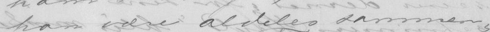han være aldeles sammen-
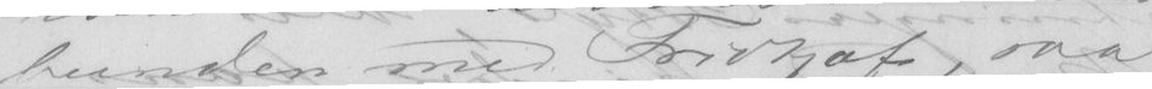bunden med Fridtjof, saa
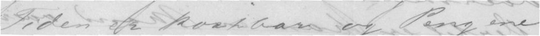Tiden er kostbar og Pengene
